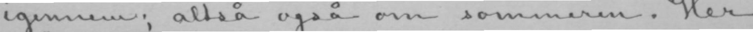igennem; altså også om sommeren. Her
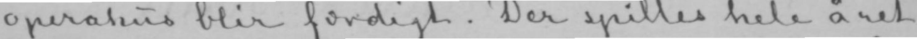operahus blir færdigt. Der spilles hele året
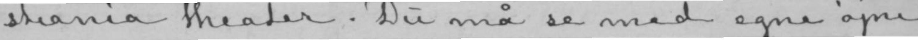stiania theater. Du må se med egne øjne
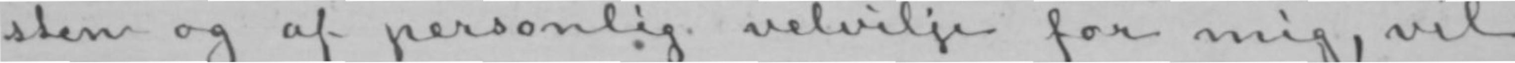sten og af personlig velvilje for mig, vil
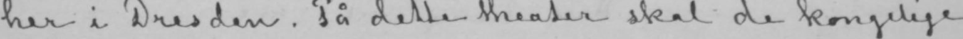her i Dresden. På dette theater skal de kongelige
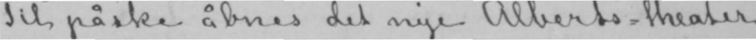Til påske åbnes det nye Alberts-theater
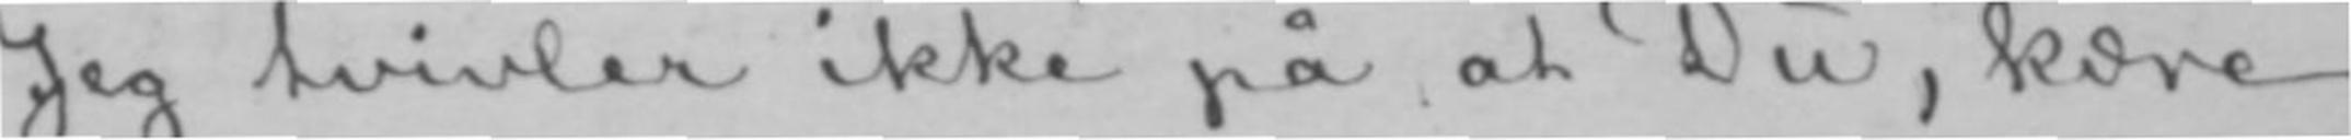Jeg tvivler ikke på at Du, kære
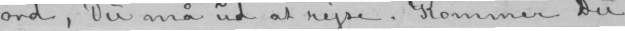ord, Du må ud at rejse. Kommer Du
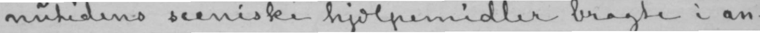nutidens sceniske hjælpemidler bragte i an-
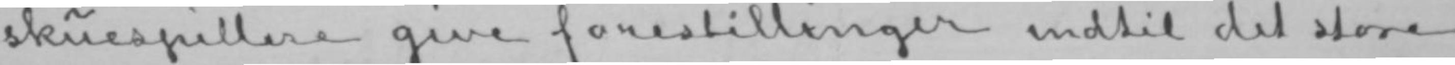skuespillere give forestillinger indtil det store
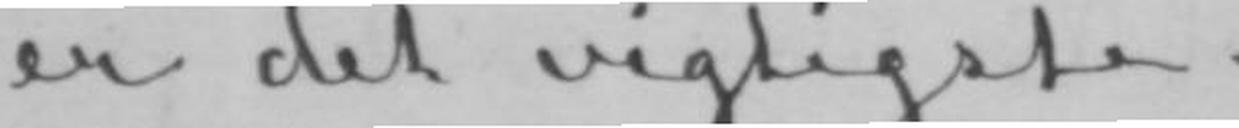er det vigtigste.
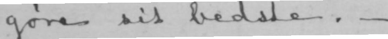gøre sit bedste.--
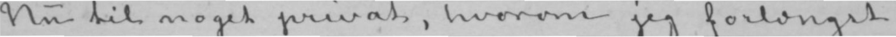Nu til noget privat, hvorom jeg forlængst
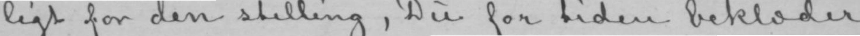ligt for den stilling, Du for tiden beklæder,
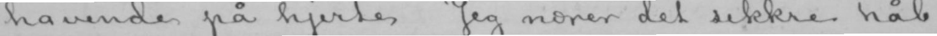havende på hjerte Jeg nærer det sikkre håb
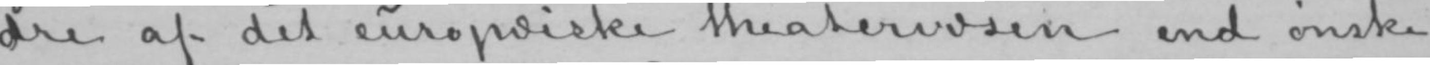dre af det europæiske theatervæsen end ønske-
